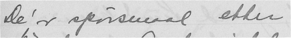De' er spørsmaal etter
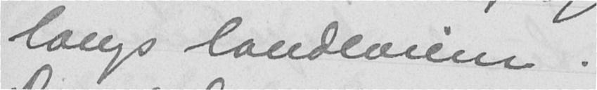langs landeveien.
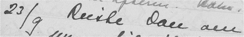23/9 Reiste Don om
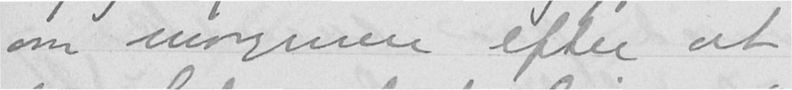om morgenen efter at
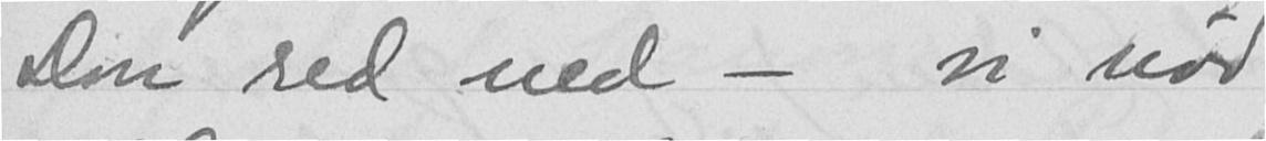Don red ned - vi nød
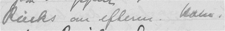Riecks om efterm. kom.
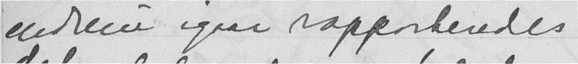endnu igaar rapporteredes
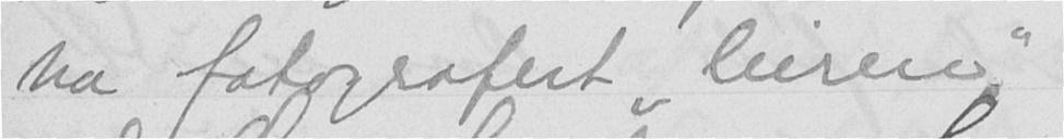ha fotografert "leiren"
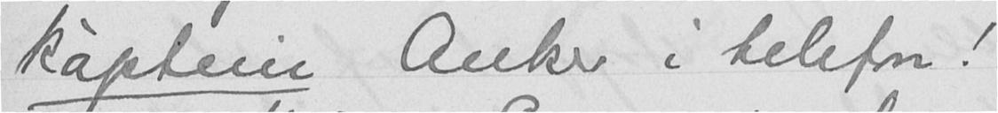kàptein Anker i telefon!
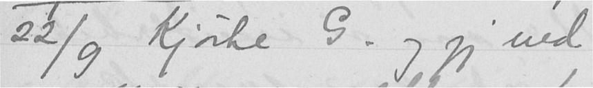22/9 Kjørte G. og jeg ned
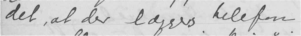det, at der lægges telefon
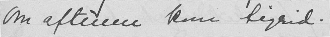Om aftenen kom Sigrid.
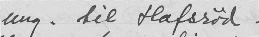mrg. til Hafsrød.
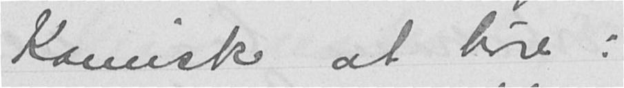Komisk at høre:
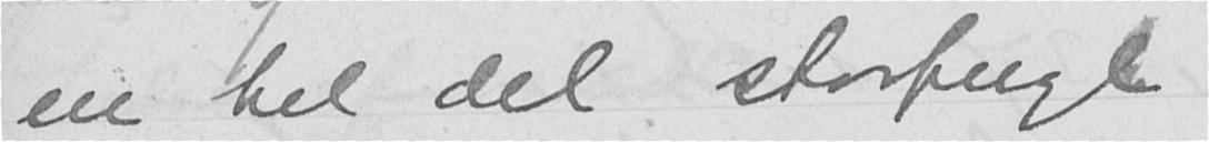en hel del storfugl
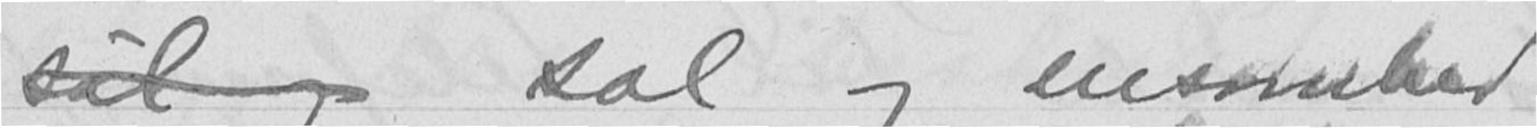sol og sol og ensomhed
